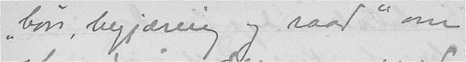"bøn, begjæring og raad" om
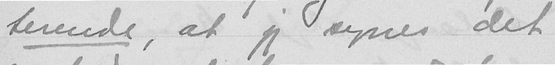terende, at jeg synes det
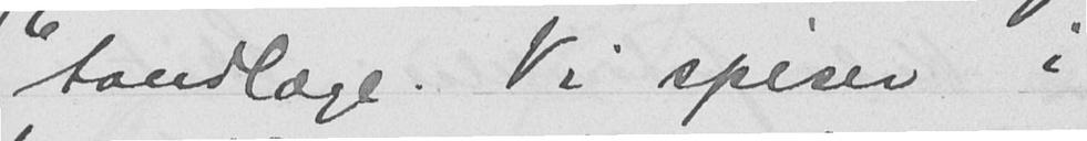tandlæge. Vi spiser i
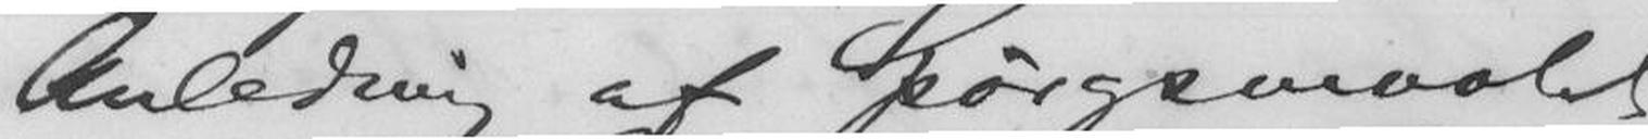Anledning af Spørgsmaalet
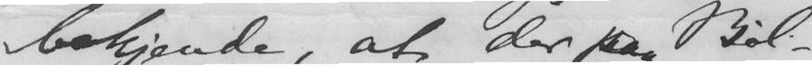bekjende, at der paa Bøl-
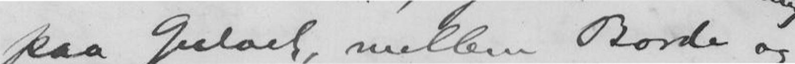paa Gulvet, mellem Borde og
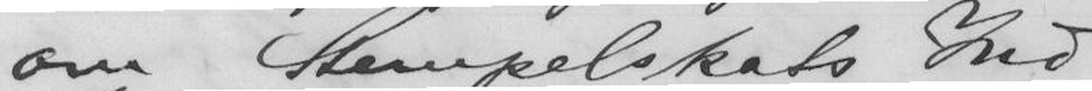om Stempelskats Ind-
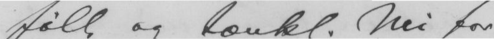følt og tænkt. Nei for
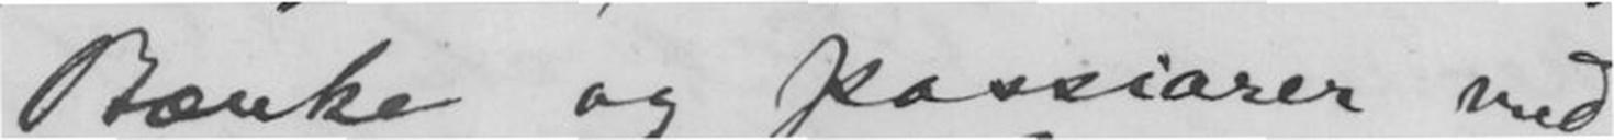Bænke og passiarer med
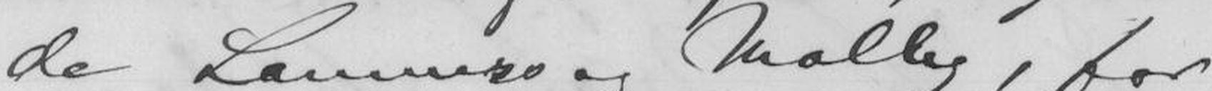de Lammers og Molly, for
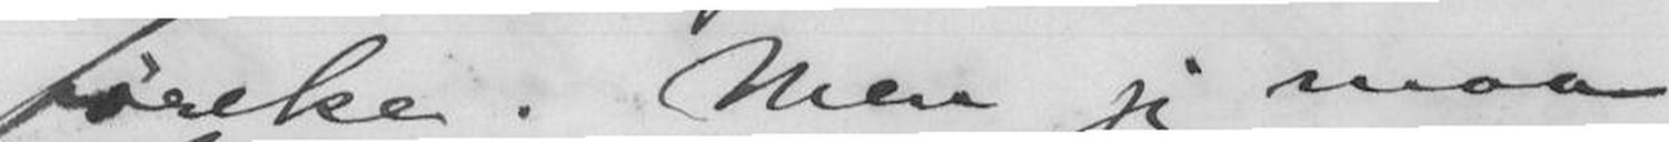førelse. Men jeg maa
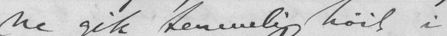ne gik temmelig høit i
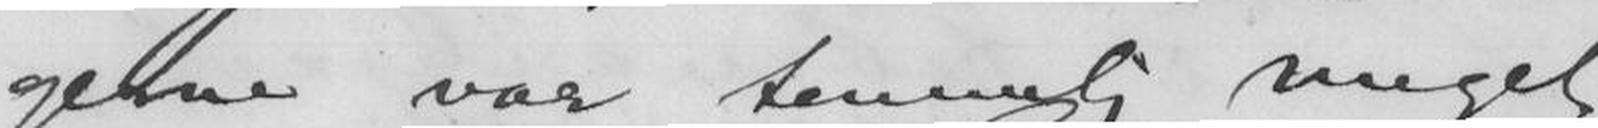gerne var temmelig meget
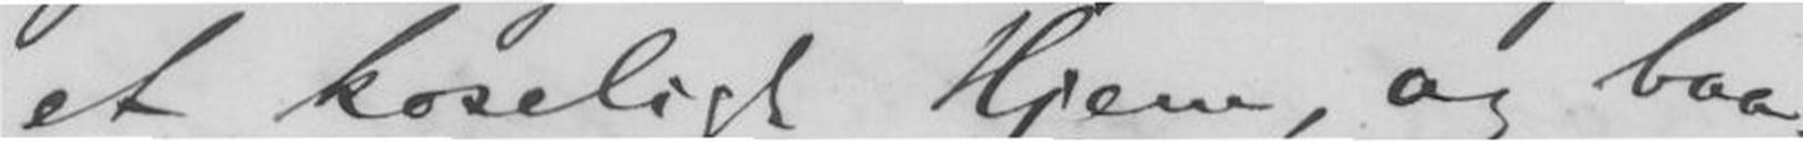et koseligt Hjem, og baa-
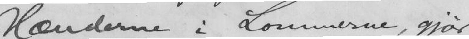Hænderne i Lommerne, gjør
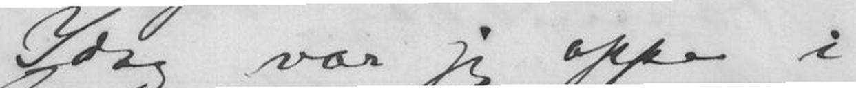Idag var jeg oppe i
